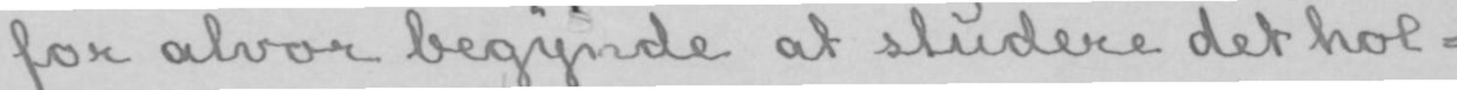for alvor begynde at studere det hol-
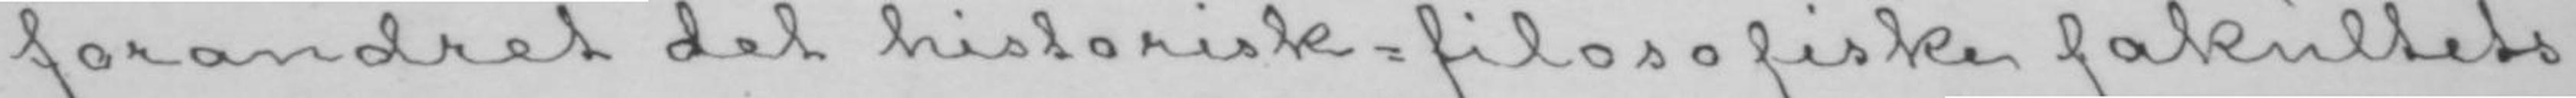forandret det historisk-filosofiske fakultets
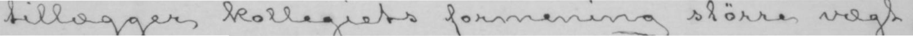tillægger kollegiets formening større vægt,
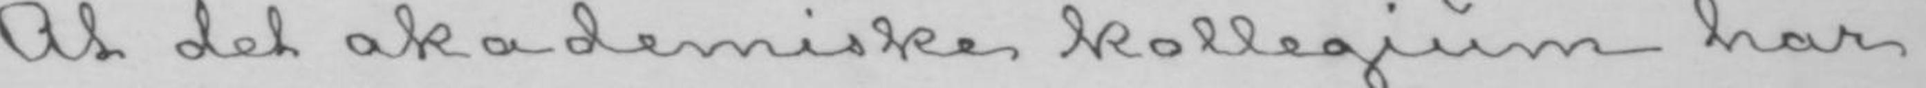At det akademiske kollegium har
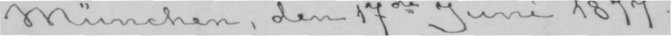München, den 17de Juni 1877.
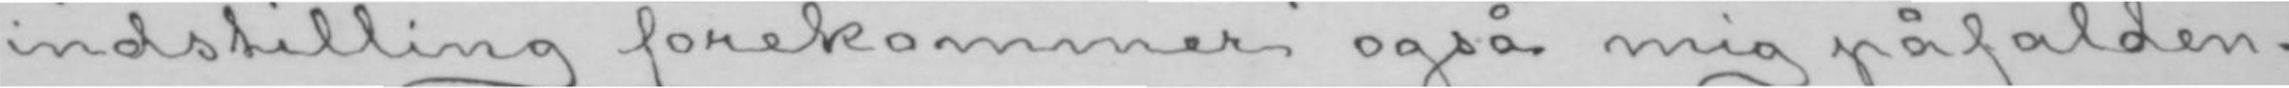indstilling forekommer også mig påfalden-
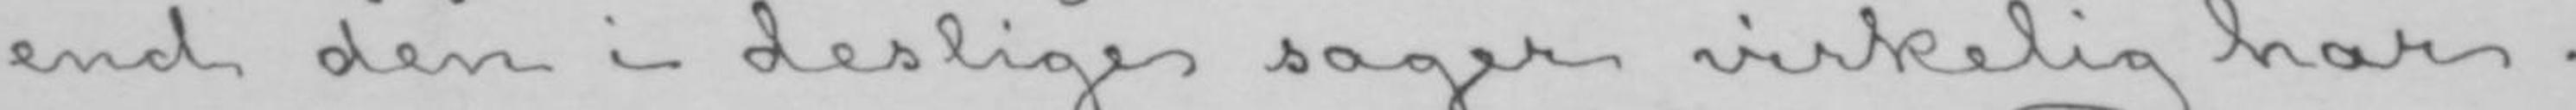end den i deslige sager virkelig har.
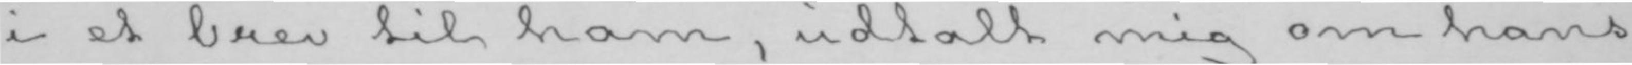i et brev til ham, udtalt mig om hans
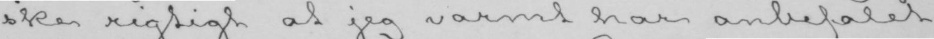ske rigtigt at jeg varmt har anbefalet
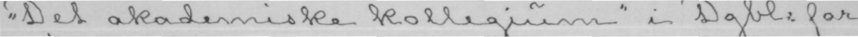«Det akademiske kollegium» i Dgbl: for
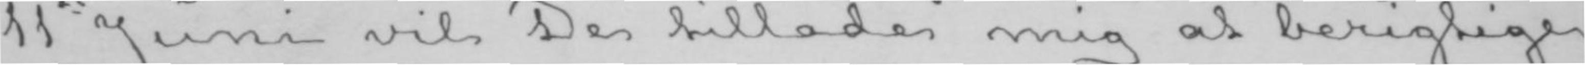11te Juni vil De tillade mig at berigtige
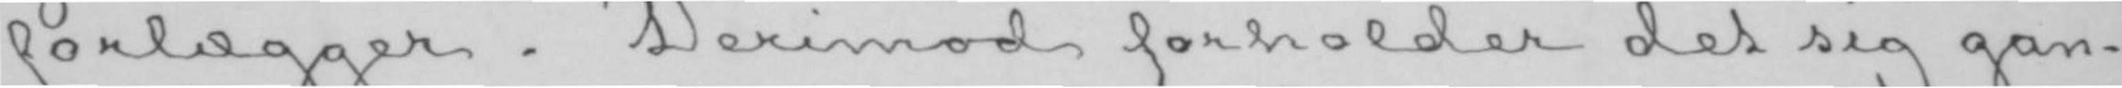forlægger. Derimod forholder det sig gan-
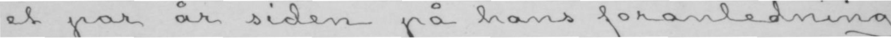et par år siden på hans foranledning,
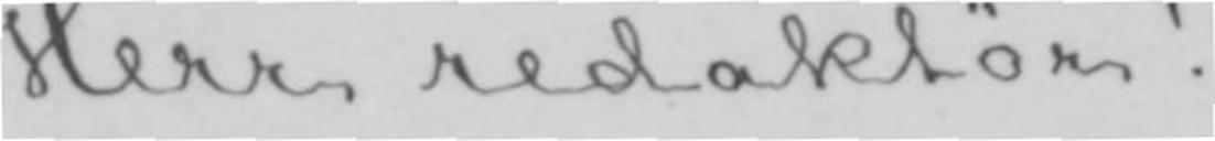Herr redaktør!
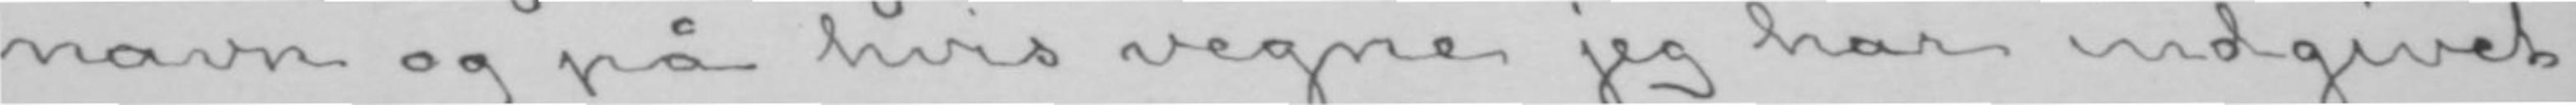navn og på hvis vegne jeg har indgivet
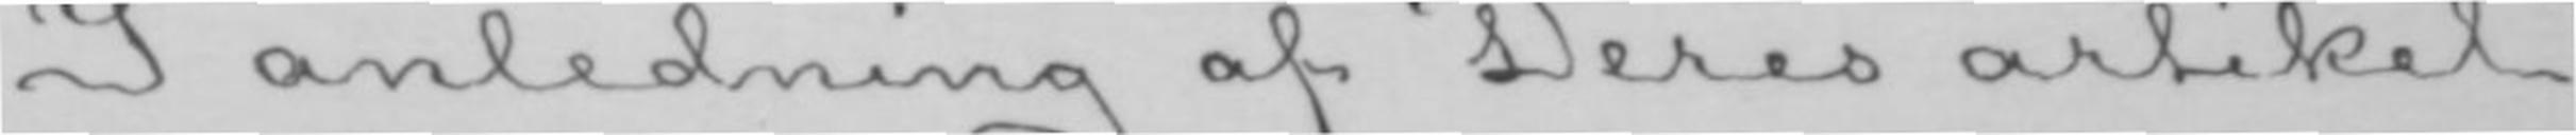I anledning af Deres artikel
